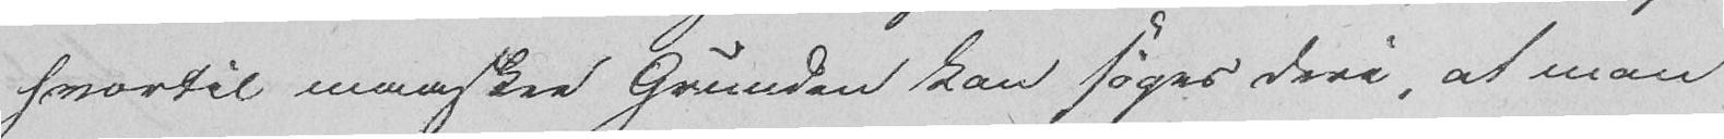hvortil maaskee Grunden kan søges deri, at man
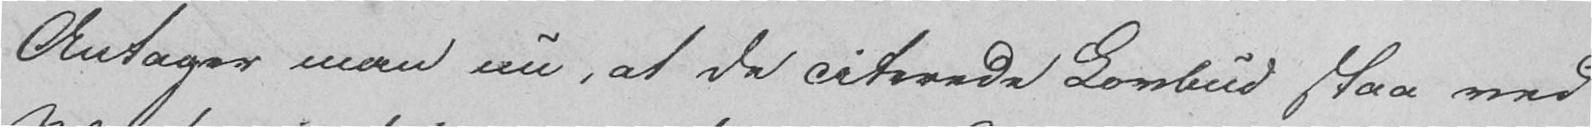Antager man nu, at de citerede Lovbud staa ved
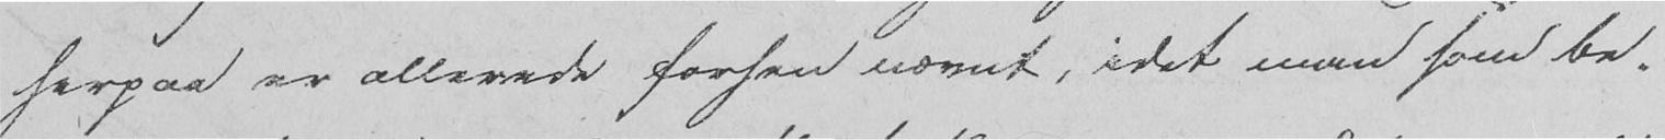herpaa er allerede forhen nævnt, idet man som be-
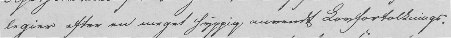legier efter en meget hyppig anvendt Lovfortolknings-
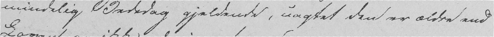mindelig Bededag gjeldende, uagtet den er ældre end
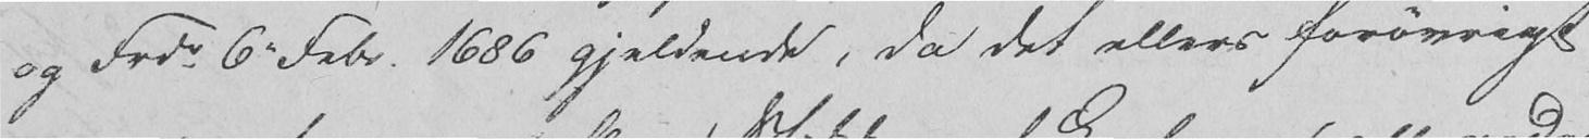og Frdn 6. Febr. 1686 gjeldende, da det ellers forøvrigt
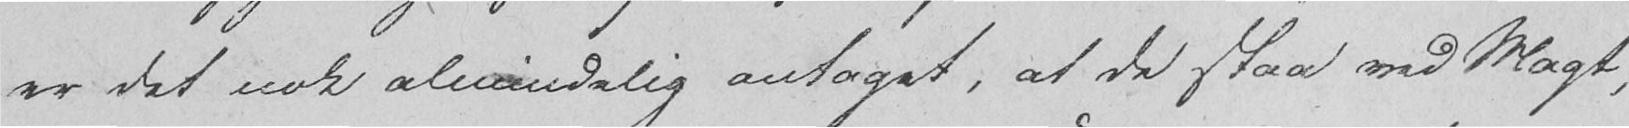er det nok almindelig antaget, at de staa ved Magt,
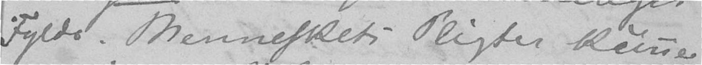Fylde. Menneskets Pligter kunne
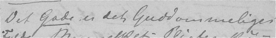Det Gode er det Guddommeliges
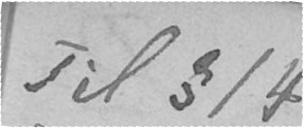Til § 14
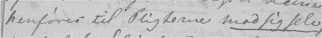henføres til Pligterne mod sig selv,
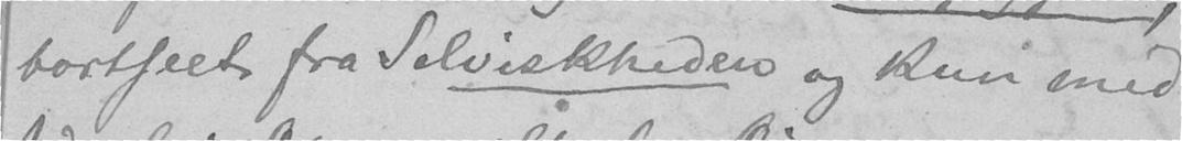bortseet fra Selviskheden og kun med
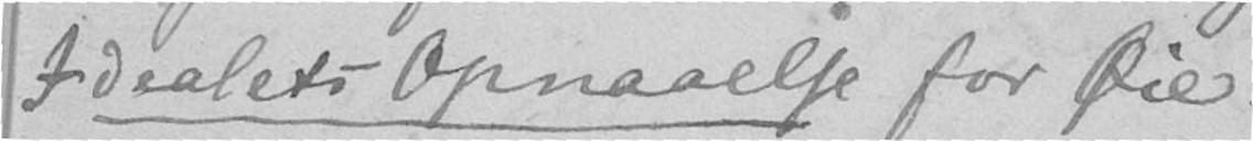Idealets Opnaaelse for Øie
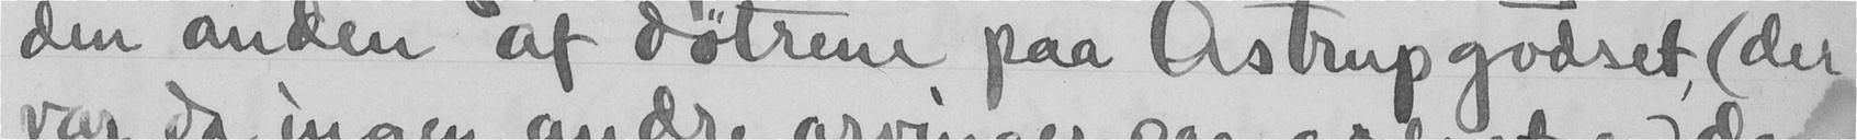den anden af døtrene paa Astrupgodset, (der
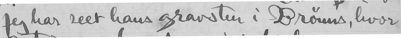Jeg har seet hans gravsten i Brøms, hvor
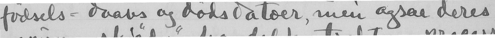fødsels - daabs og dødsdatoer, men ogsaa deres
